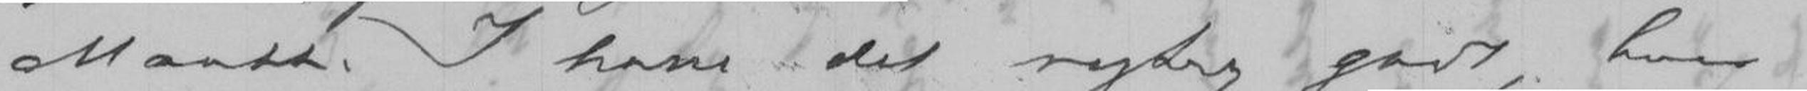Maatte I have det rigtig godt, hvor I
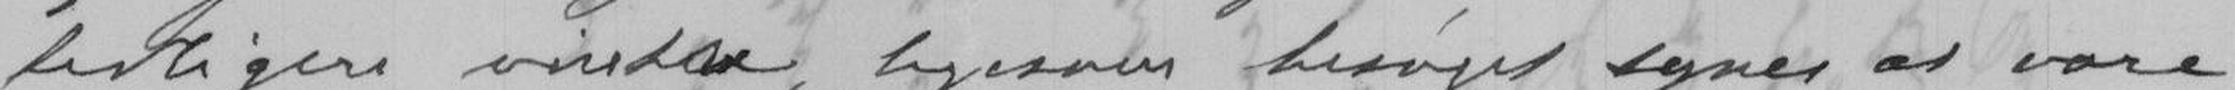tidligere vintre, ligesom besøget synes at være
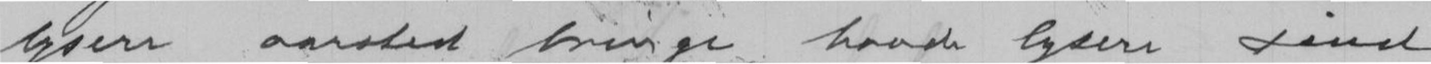lysere aarstid bringe baade lysere sind
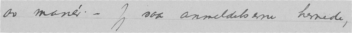av manèr. – Jeg saa anmeldelserne hernede,
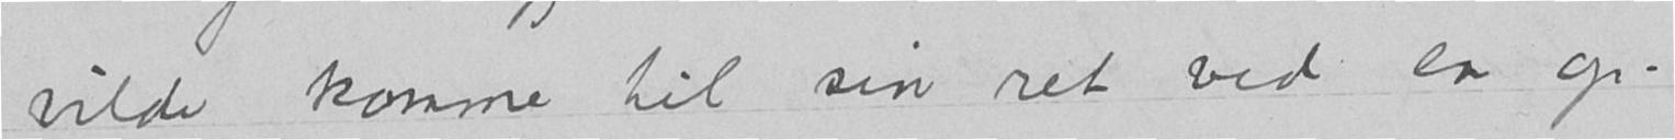vilde komme til sin ret ved en op-
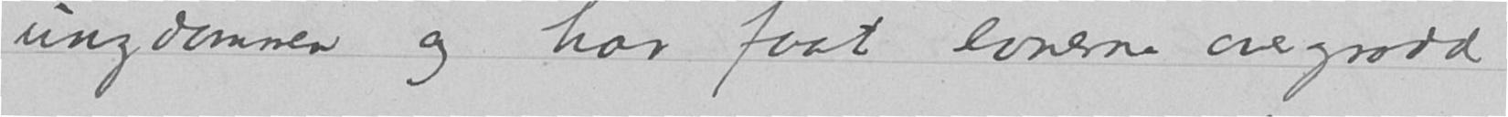ungdommen og har faat evnerne overgrodd
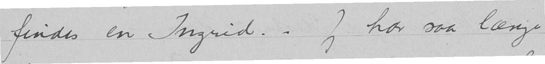findes en Ingrid. – Jeg har saa længe
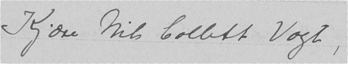Kjære Nils Collett Vogt,
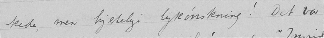kede, men hjertelige lykønskning. Det var
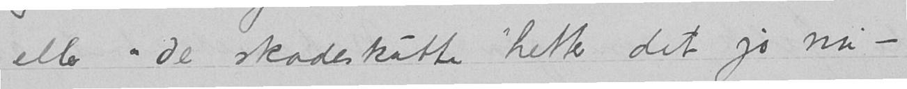eller «De skadeskutte" hetter det jo nu -
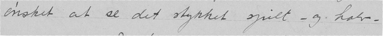ønsket at se det stykket spilt – og halv-
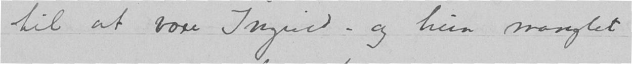til at være Ingrid – og hun manglet
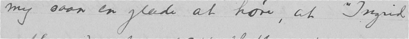mig saan en glæde at høre, at "Ingrid"
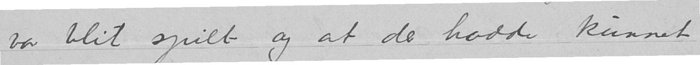var blit spilt og at der hadde kunnet
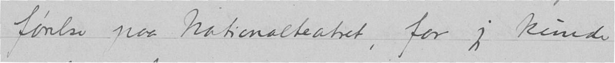førelse paa Nationalteatret, for jeg kunde
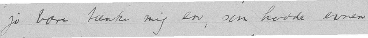jo bare tænke mig en, som hadde evnen
